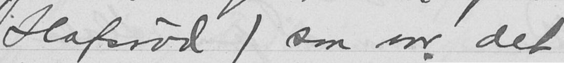Hafsrød ) saa var det
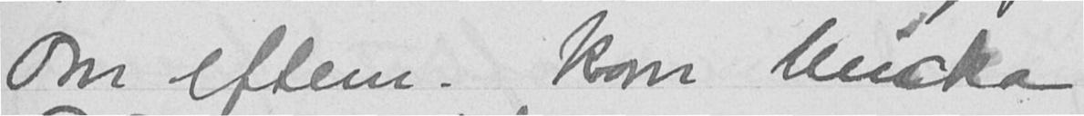Om efterm. kom Micka
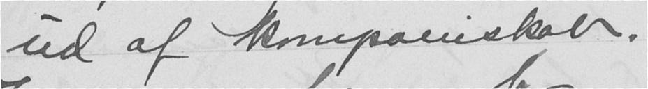ud af kompaniskab.
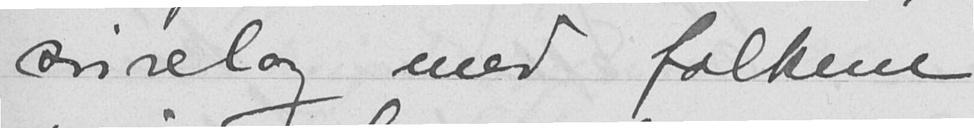svirelag med folkene
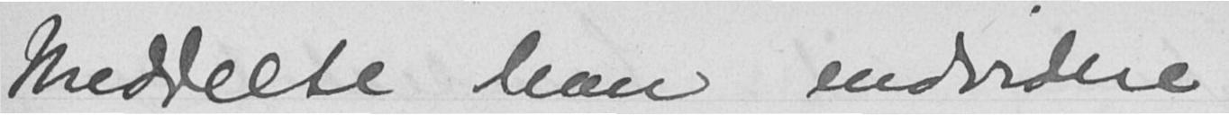Meddelte han endvidere
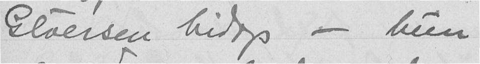Gløersen hidop - hun
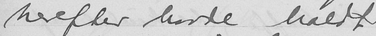herefter havde holdt
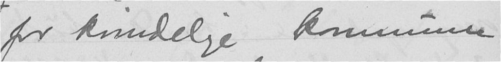for kvindelige kommune
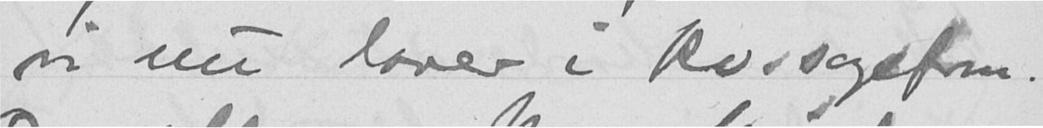vi nu lover i kv.sagsforn.
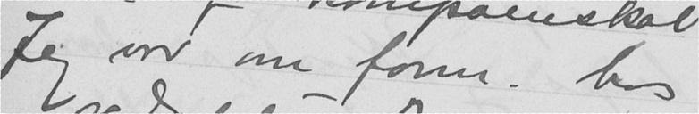Jeg var om form. hos
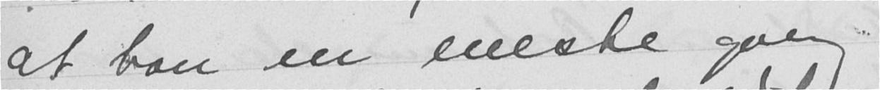at han en eneste gang
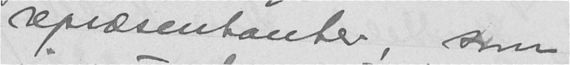repræsentanter, som
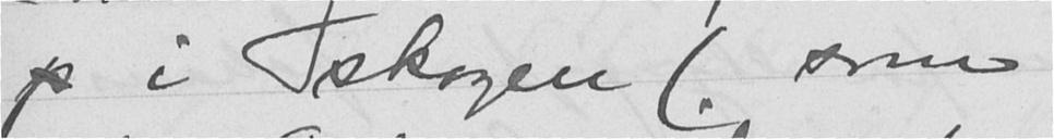p i skogen (som
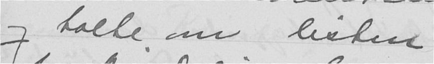og talte om listen
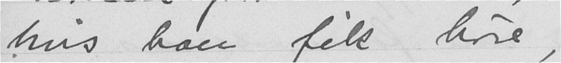hvis han fik høre,
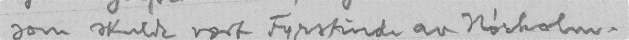som skulde vært Fyrstinde av Nørholm.
